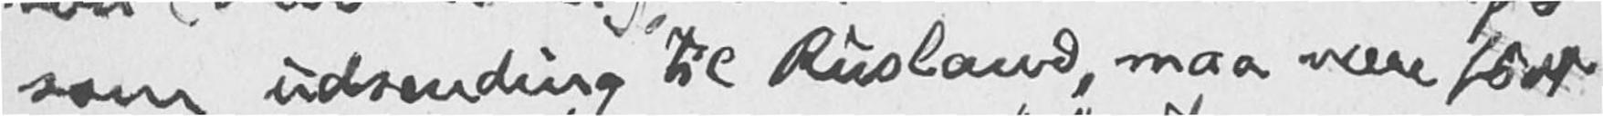som udsending til Rusland, maa være født
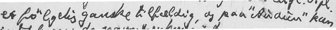er følgelig ganske tilfældig, og paa "Audun" kan
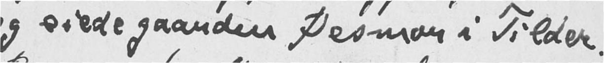og eiede gaarden Þesmon i Tilder.
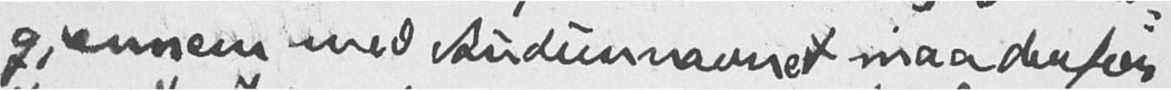gjennem med Audunnavnet maa derfor
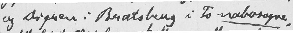og Digren i Bratsberg i to nabosogne,
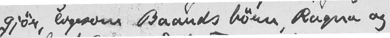gjør, ligesom Baards børn, Ragna og
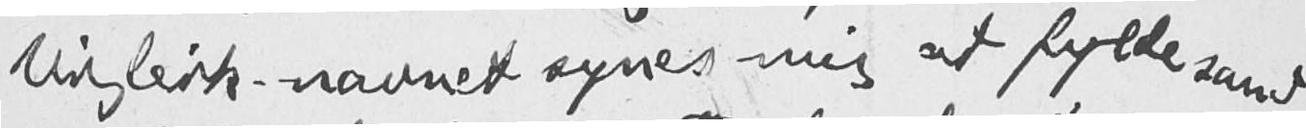Vigleik-navnet synes mig at fylde sand-
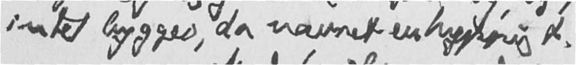intet bygges, da navnet er hyppigt.
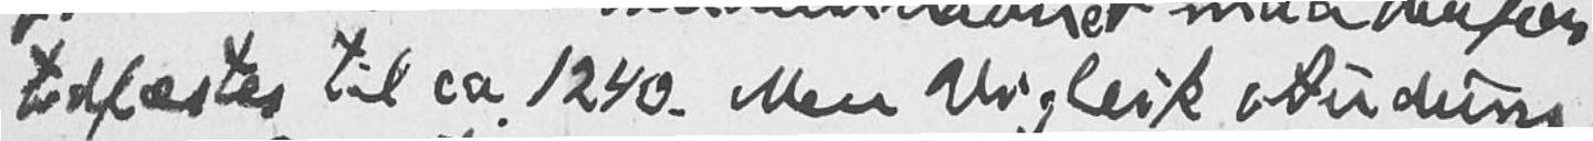tidfæstes til ca 1240. Men Vigleik Auduns-
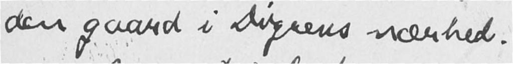den gaard i Digrens nærhed.
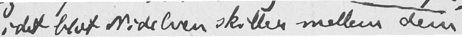i det blot Nidelven skiller mellem dem.
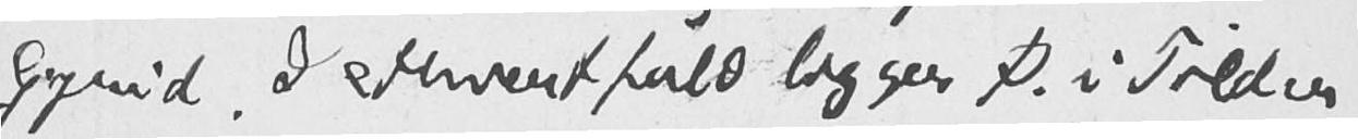Gyrid. I ethvert fald ligger Þ. i Tilder
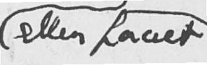eller faaet
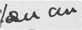en an-
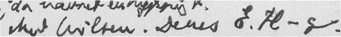Med hilsen. Deres E. H-g.
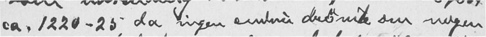ca. 1220-25, da ingen endnu drømte om nogen
